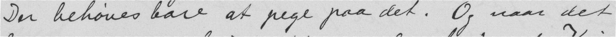Der behøves bare at pege paa det. Og naar det
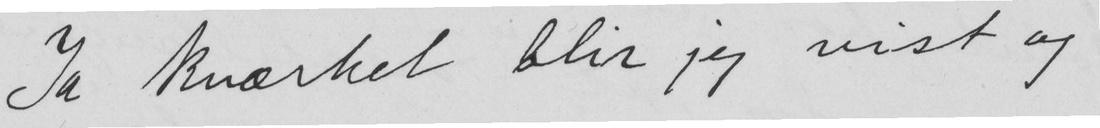Ja kværket blir jeg vist og
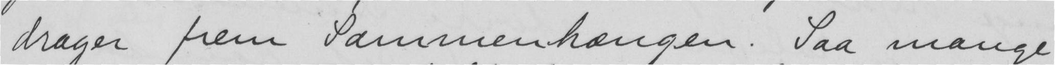drager frem Sammenhængen. Saa mange
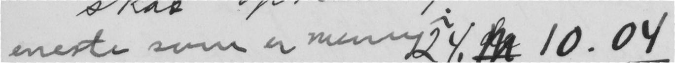eneste som er mening 24. 10.04
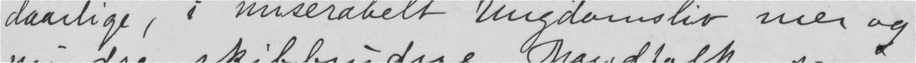daarlige, i miserabelt Ungdomsliv mer og
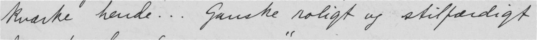kværke hende.. Ganske roligt og stilfærdigt
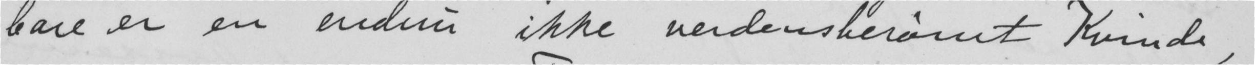bare er en endnu ikke verdensberømt Kvinde,
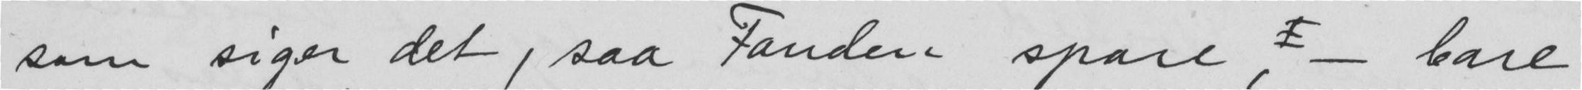som siger det, saa Fanden spare, - bare
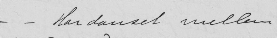— — Har danset mellem
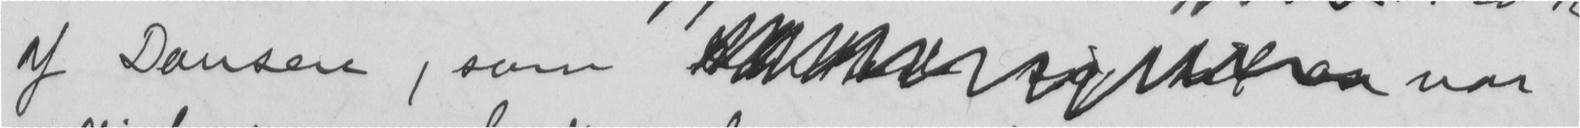af Dansere, som  var
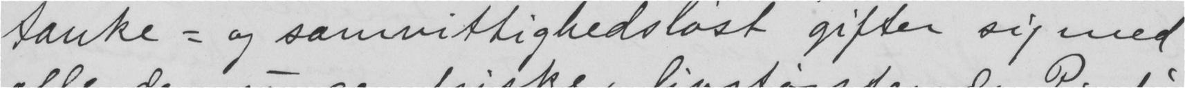tanke - og samvittighedsløst gifter sig med
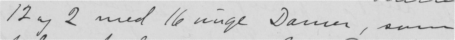12 og 2 med 16 unge Damer, som
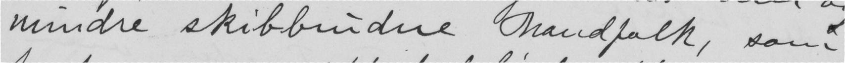mindre skibbundne Mandfolk, som
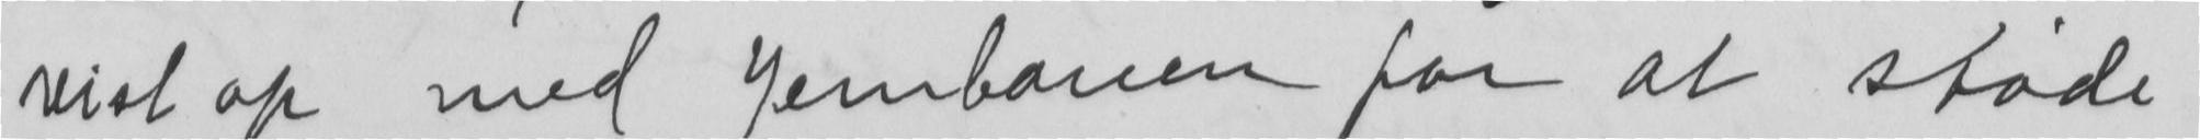vist op med Jernbanen for at støde
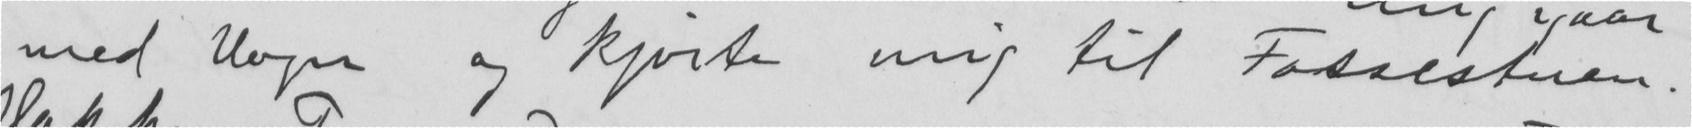med Vogn og kjørte mig til Fossestuen.
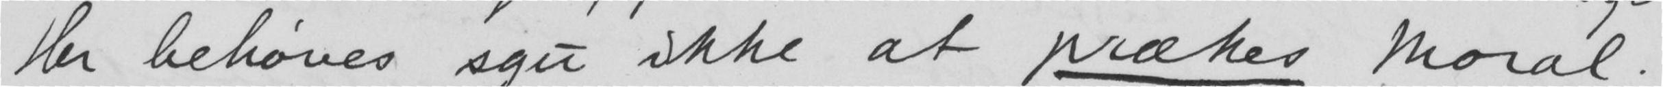Her behøves sgu ikke at prækes Moral.
